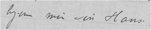hjem min søn Hans.
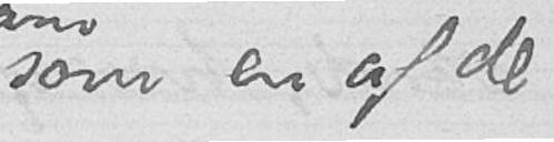som en af de
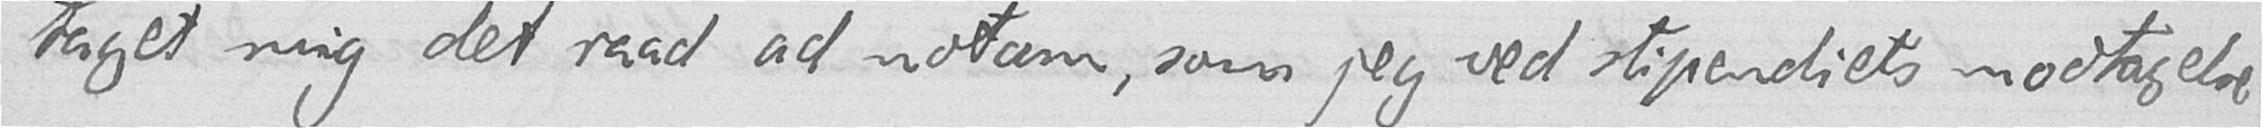taget mig det raad ad notam, som jeg ved stipendiets modtagelse
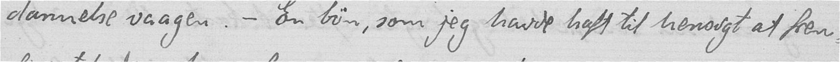dannelse vaagen. - En bøn, som jeg havde haft til hensigt at frem-
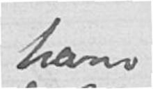ham
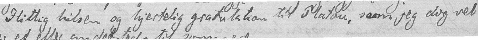Flittig hilsen og hjertelig gratulation til Platon, som jeg dog vel
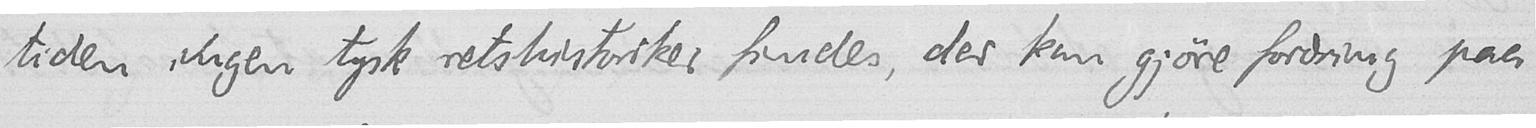tiden ingen tysk retshistoriker findes, der kan gjøre fordring paa
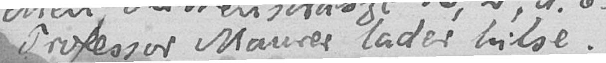Professor Maurer lader hilse.
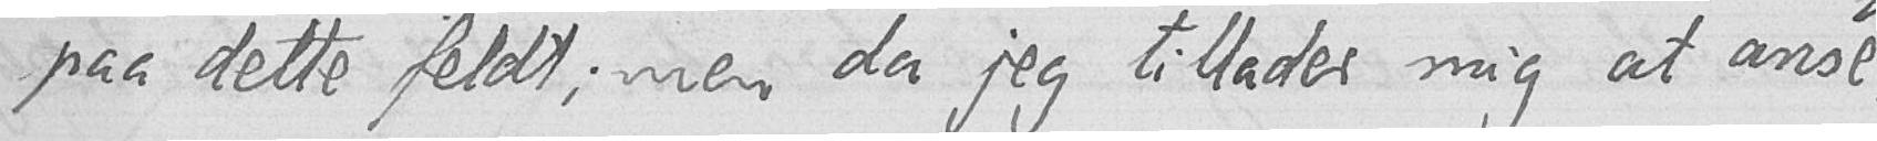paa dette feldt; men da jeg tillader mig at anse
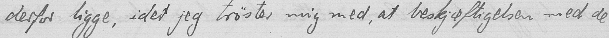derfor ligge, idet jeg trøster mig med, at beskjæftigelsen med de
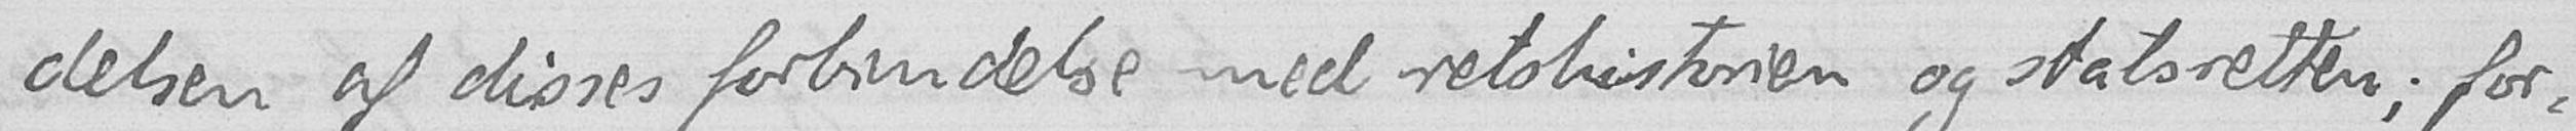delsen af disses forbindelse med retshistorien og statsretten; for-
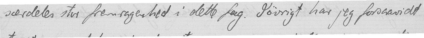særdeles stor fremragenhed i dette fag. Iøvrigt har jeg forsaavidt
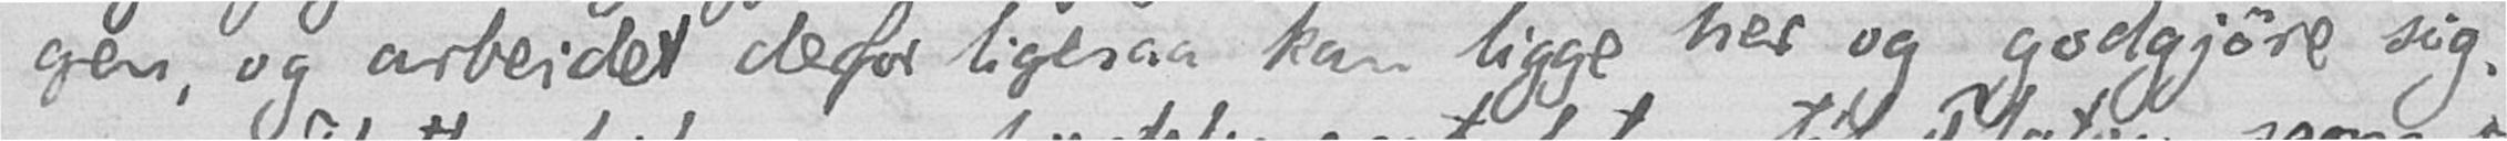gen, og arbeidet derfor ligesaa kan ligge her og godgjøre sig.
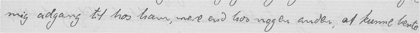mig adgang til hos ham, mere end hos nogen anden, at kunne hente
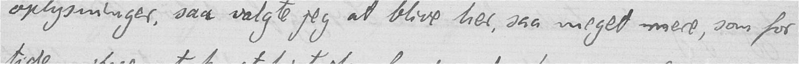oplysninger, saa valgte jeg at blive her, saa meget mere, som for
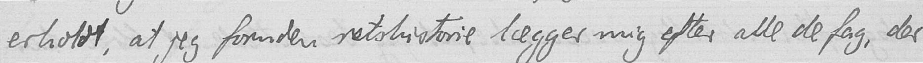erholdt, at jeg foruden retshistorie lægger mig efter alle de fag, der
